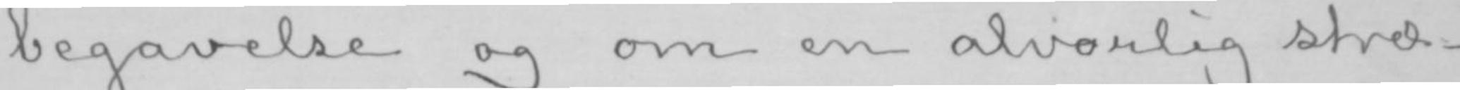begavelse og om en alvorlig stræ-
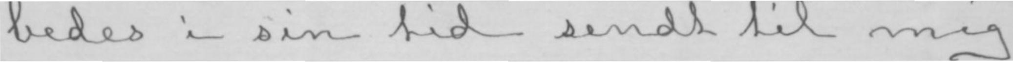bedes i sin tid sendt til mig,
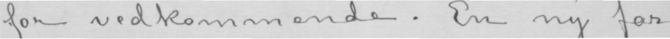for vedkommende. En ny for-
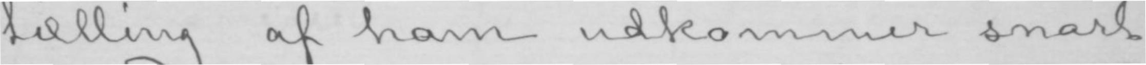tælling af ham udkommer snart
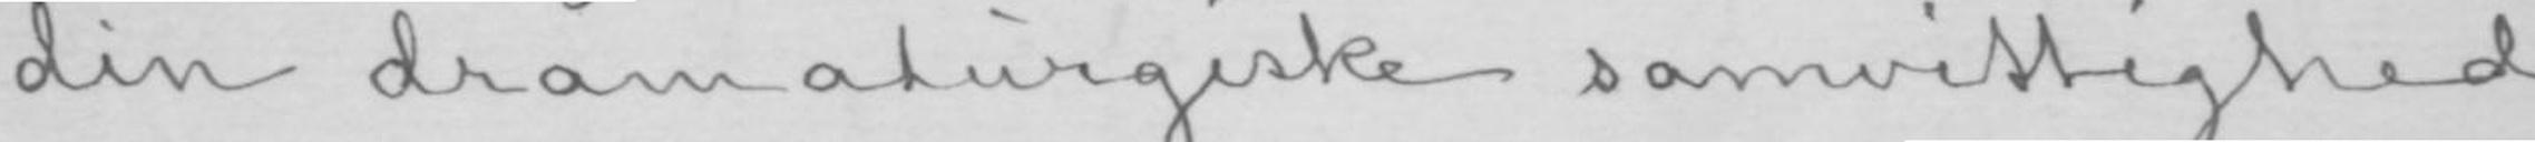din dramaturgiske samvittighed
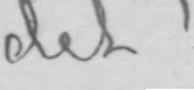det!
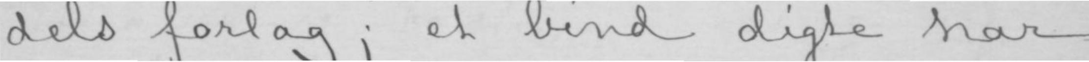dels forlag; et bind digte har
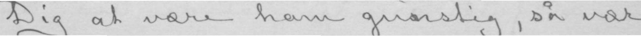Dig at være ham gusnstig, så vær
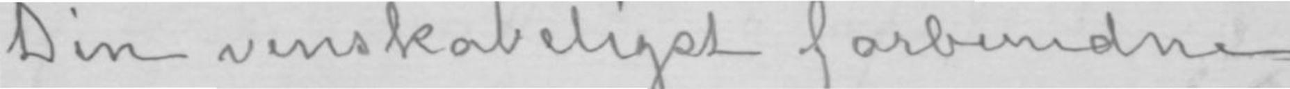Din venskabeligst forbundne
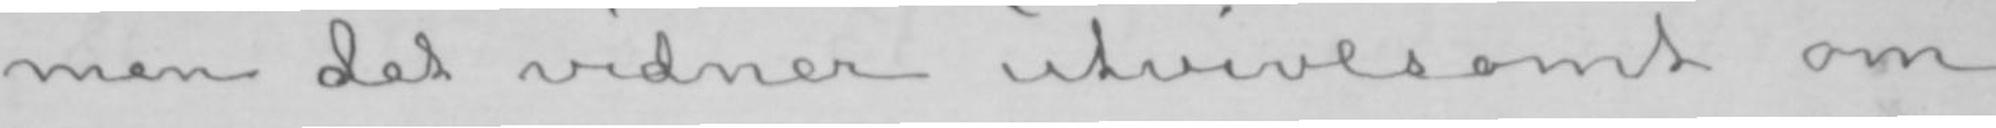men det vidner utvivlsomt om
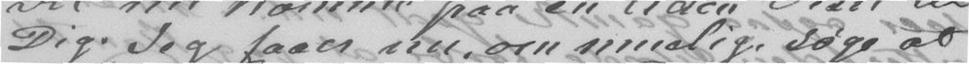Dig. Jeg faaer nu, om muelig søge at
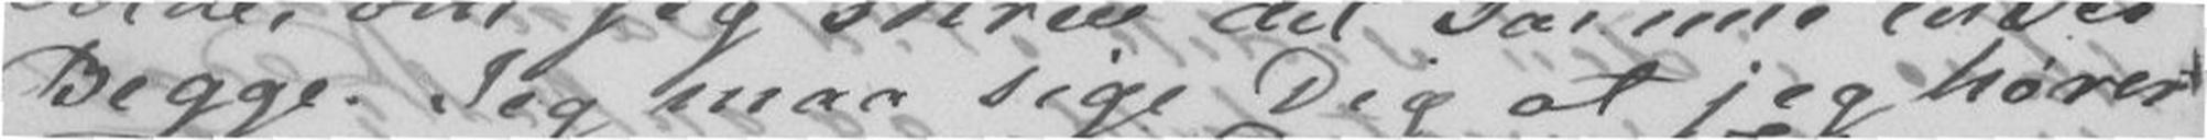Begge. Jeg maa sige Dig at jeg hører
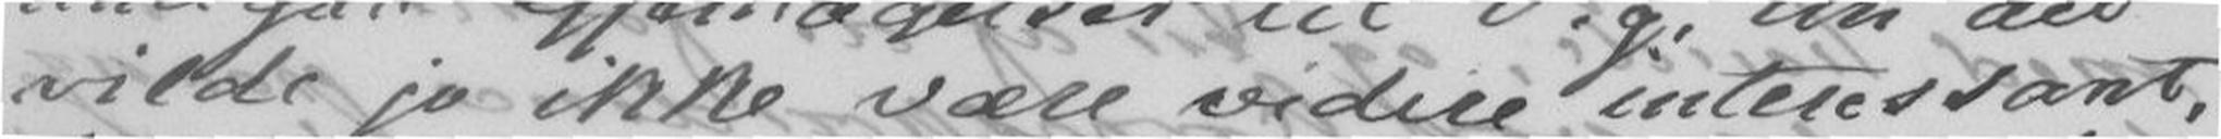vilde jo ikke være videre interessant,
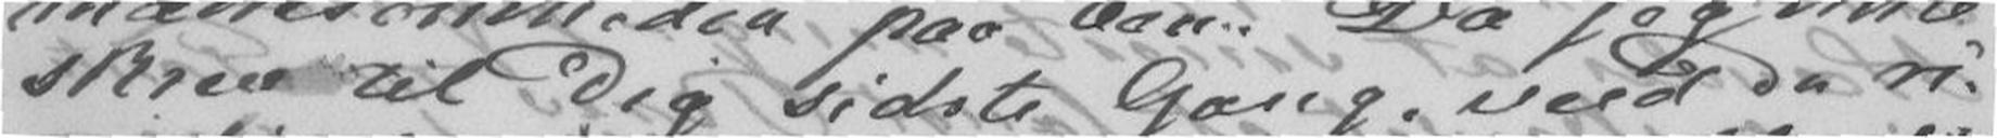skrev til dig sidste Gang, veed Du ri-
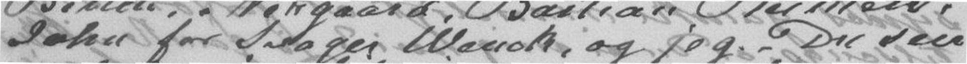John for Svoger Wenck og jeg. Du seer
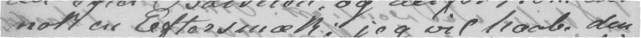nok en Eftersmæk; jeg vil haabe den
